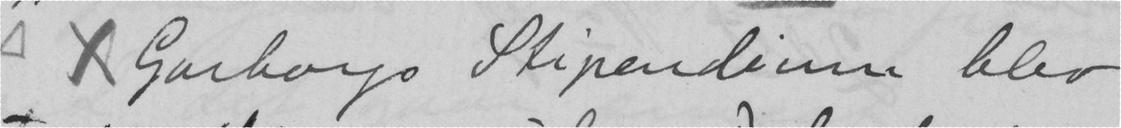x Garborgs Stipendium blev
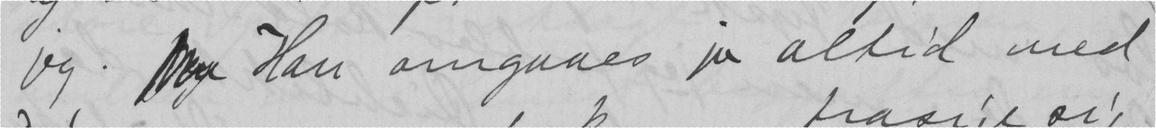jeg.  Han omgaaes jo altid med
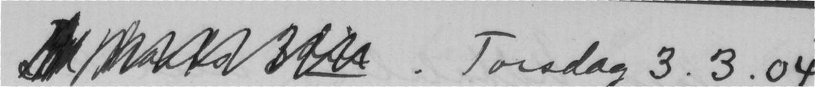. Torsdag 3.3.04
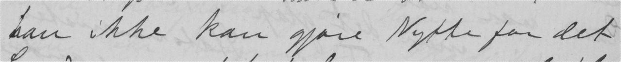han ikke kan gjøre Nytte for det
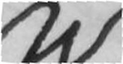W
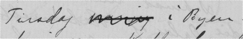Tirsdag  i Byen.
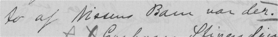to af Nissens Barn var der.
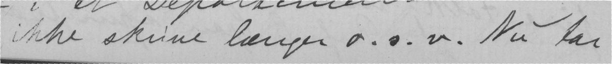ikke skrive længer o. s. v. Nu tar
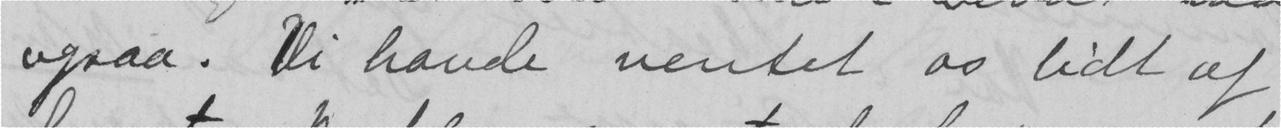ogsaa. Vi havde ventet os lidt af
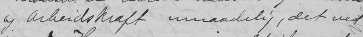og Arbeidskraft umaadelig, det ved
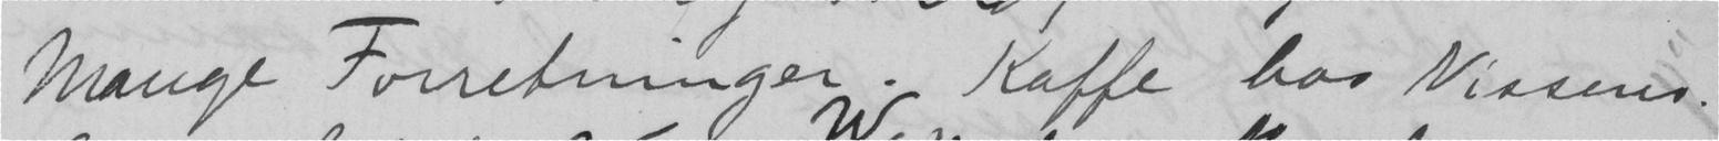Mange Forretninger. Kaffe hos Nissens.
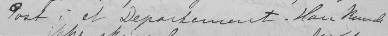Post i et Departement. Han kunde
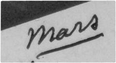mars
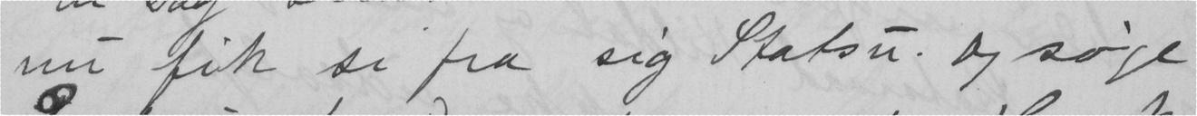nu fik si fra sig Statsu. og søge
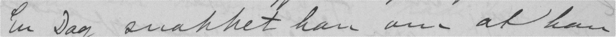en Dag snakket han om at han
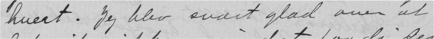hvert. Jeg blev svært glad over at
